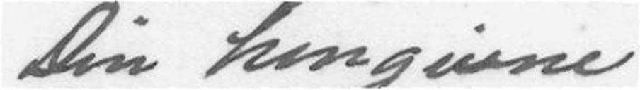Din hengivne
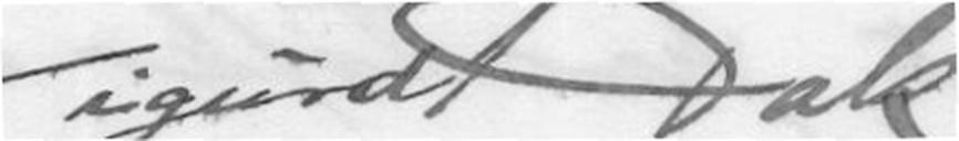Sigurd Hals
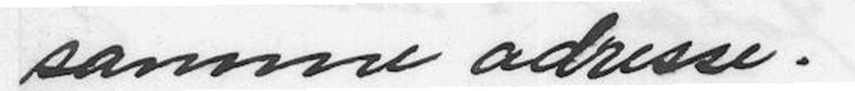samme adresse.
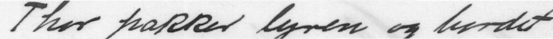Thor pakker lyren og bordet
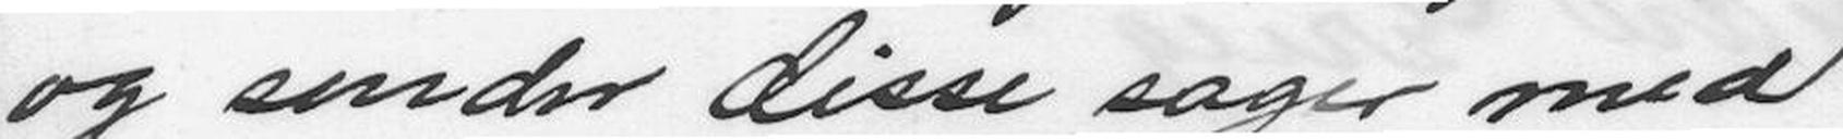og sender disse sager med
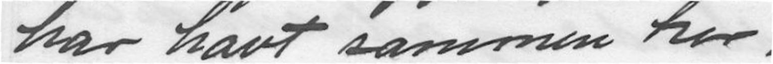har havt sammen her.
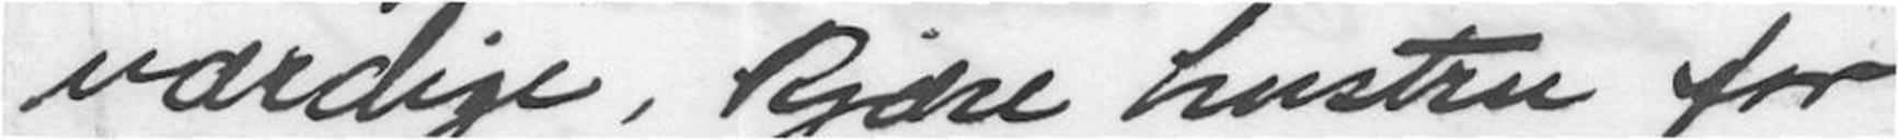værdige, kjære hustru for
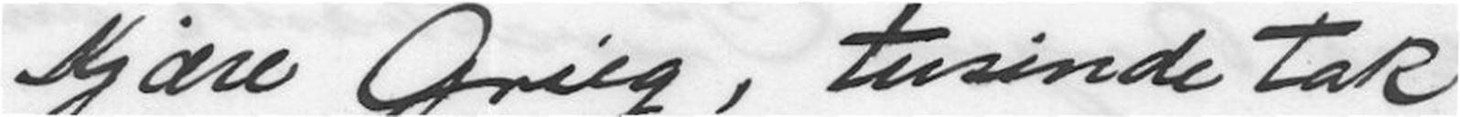Kjære Grieg, tusinde tak
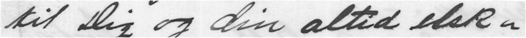til Dig og din altid elsk-
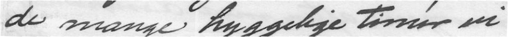de mange hyggelige timer vi
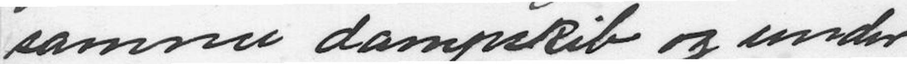samme dampskib og under
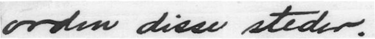orden disse steder.
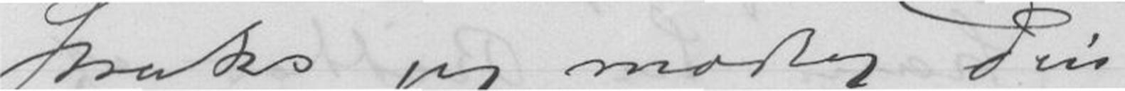Straks jeg modtog din
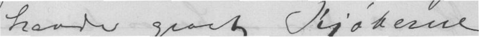havde givet Kjøberne
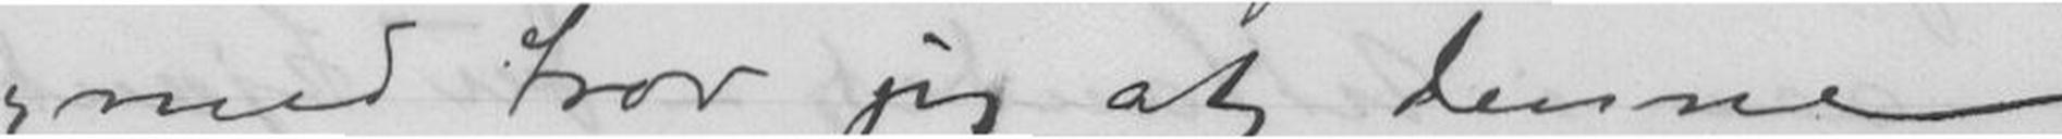med tror jeg at denne
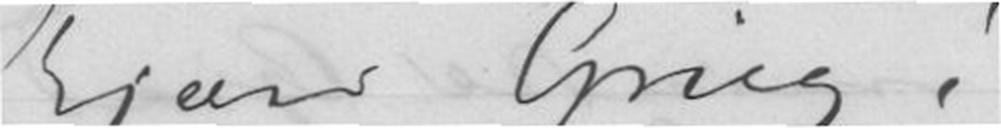Kjære Grieg!
